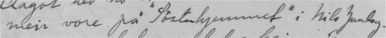meir vore på "Sösterhjemmet" i Nils Junlag.
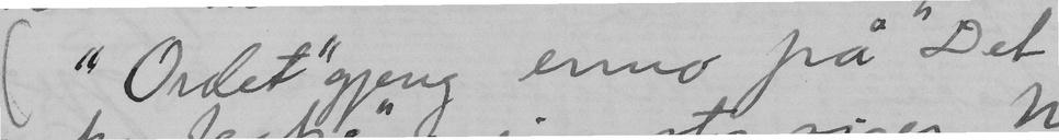"Ordet" gjeng enno på "Det
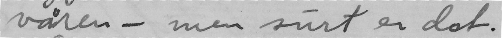våren – men surt er det.
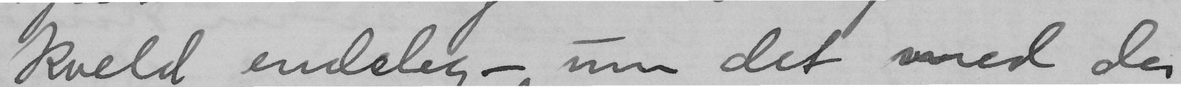kveld endeleg – um det med dei
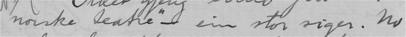norske teatre" – ei stor siger. No
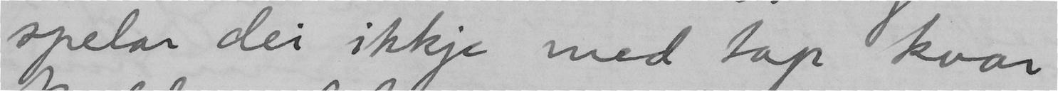spelar dei ikkje med tap kvar
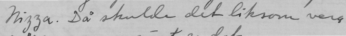Nizza. Då skulde det liksom vera
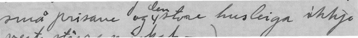små prisane og den store husleiga ikkje
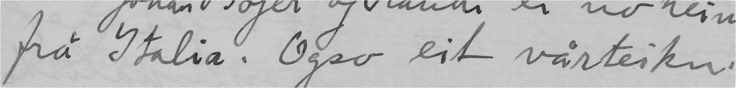frå Italia. Også eit vårteikn.
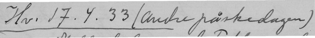Hv. 17.4.33 (andre påskedagen)
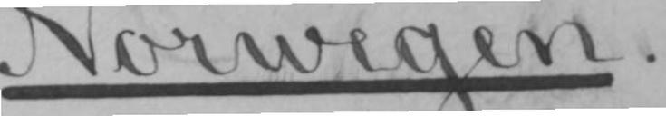Norwegen.
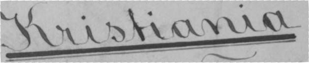Kristiania.
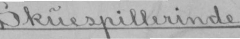Skuespillerinde
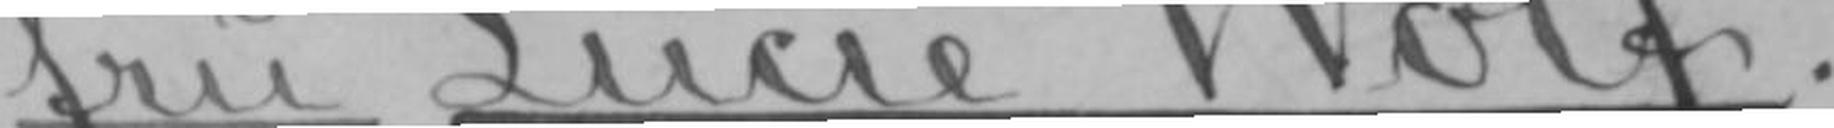fru Lucie Wolf.
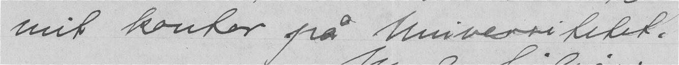mit kontor på Universititet.
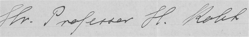Hr. Professor H. Koht
